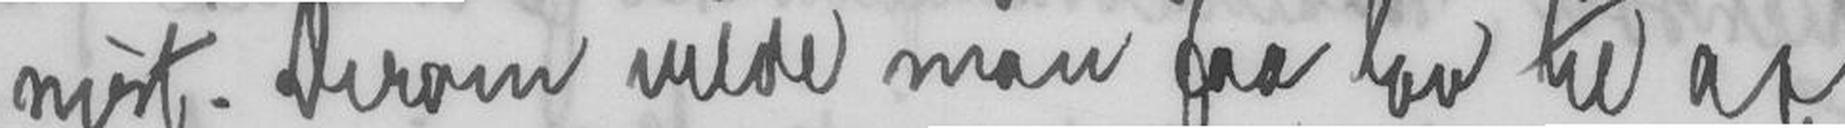nist. Derom vilde man faa lov til at
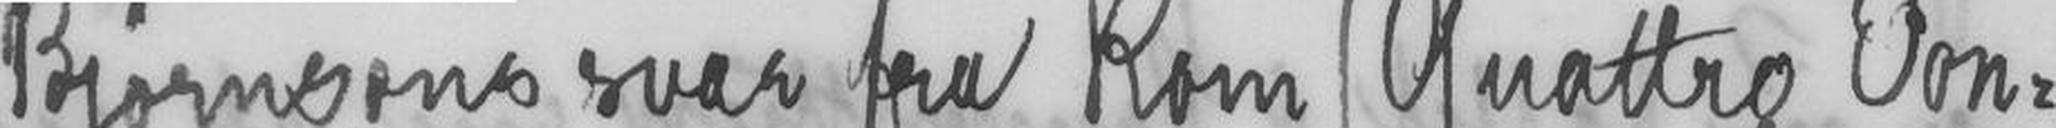Bjørnsons svar fra Rom (Quattro Ton-
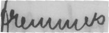fremmes
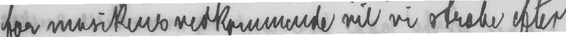for musikersvedkommende vil vi stræbe efter
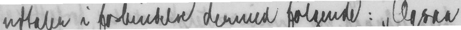udtaler i forbindelse dermed følgende: Ogsaa
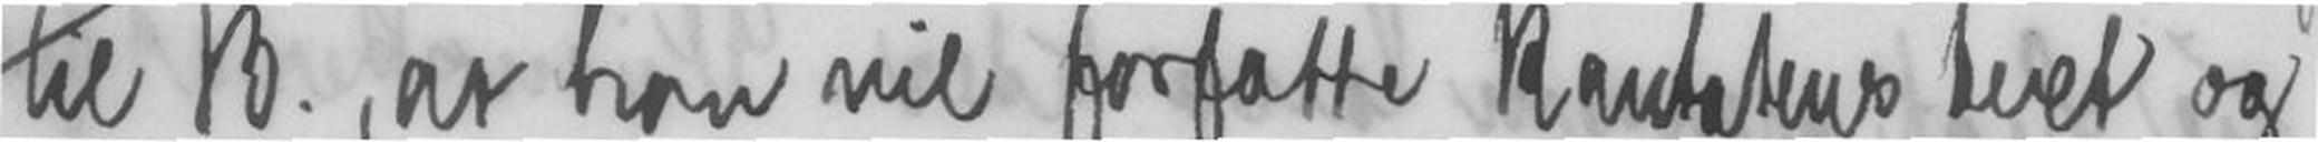til B., at han vil forfatte kantatens text og
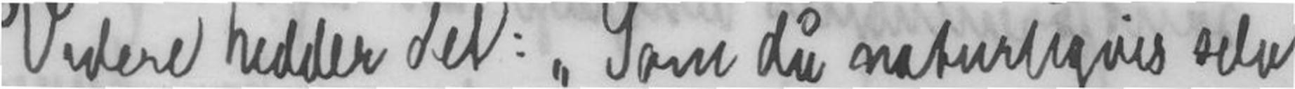Videre hedder det: Som du naturligvis selv
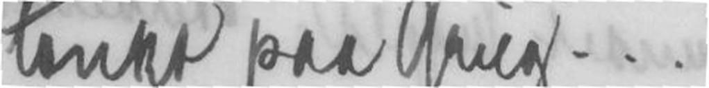tænkt paa Grieg...
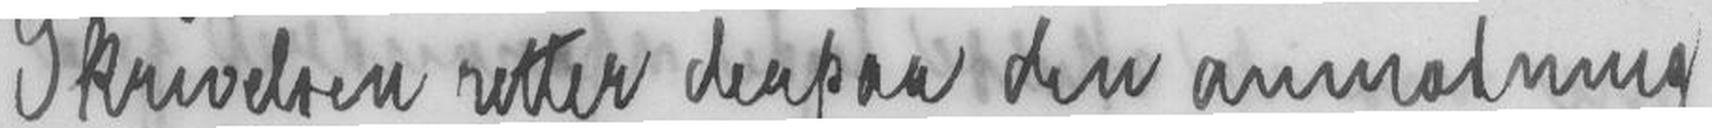Skrivelsen retter derpaa den anmodning
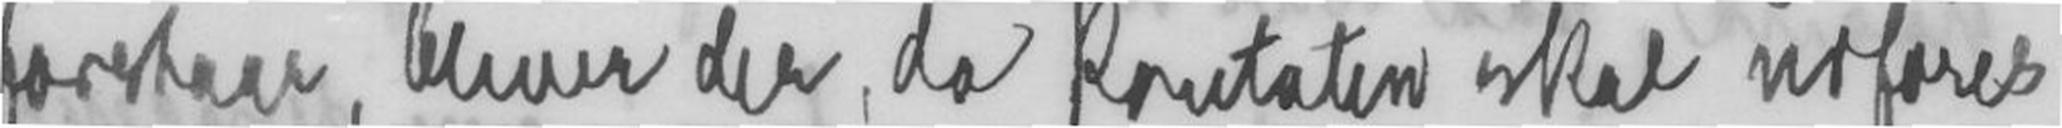forstaar, bliver der, da kantaten skal udføres
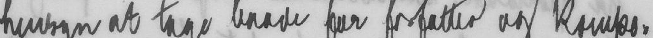hensyn at tage baade for forfatter og kompo-
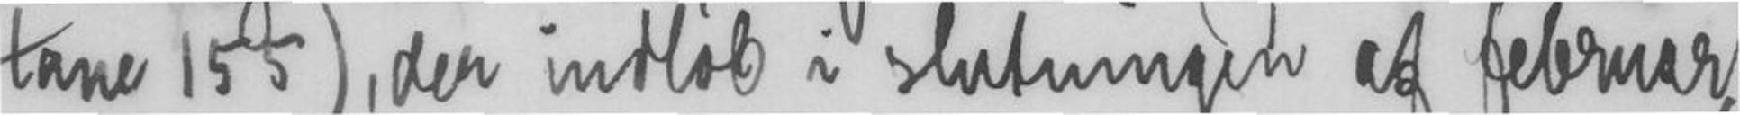tane 155), der indløb i slutningen af februar,
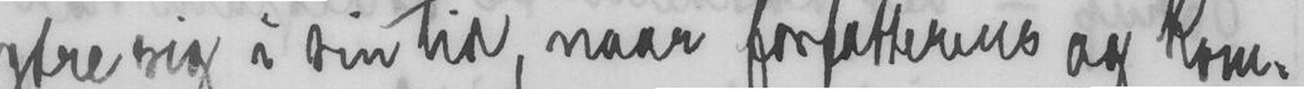ytre sig i sin tid, naar forfatterens og kom-
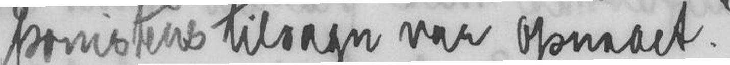ponistens tilsagn var opnaaet.
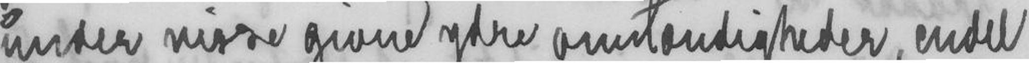under visse givne ydre omstændigheder, endel
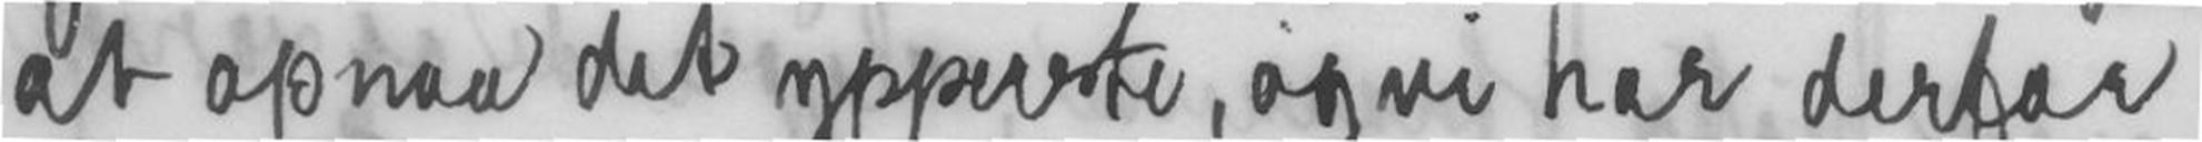at opnaa det ypperste, og vi har derfor
